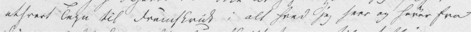ethvert Tegn til Fremskridt i alt hvad jeg seer og hører fra
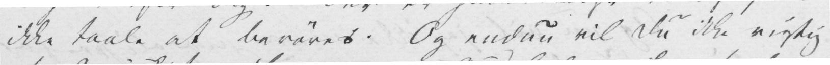ikke taale at berøres. Og endnu vil Du ikke rigtig
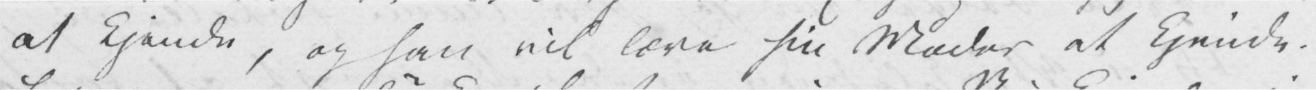at kjende, og han vil lære sin Moder at kjende.
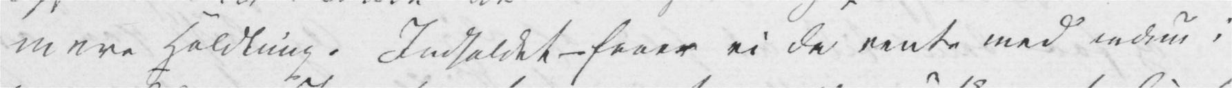mere Holdning. Indholdet – faaer vi da vente med endnu i
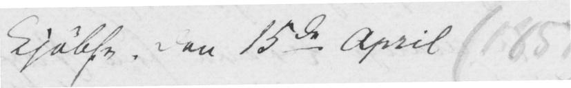Kjøbhn. Den 15de April
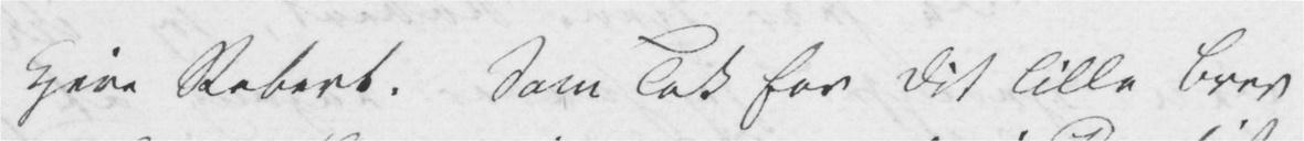Kjere Robert. Som Tak for Dit lille Brev
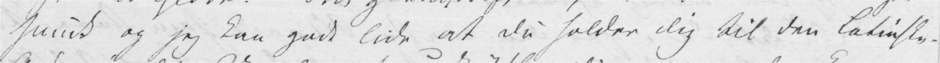smuk og jeg kan godt lide at Du holder Dig til den Latinske.
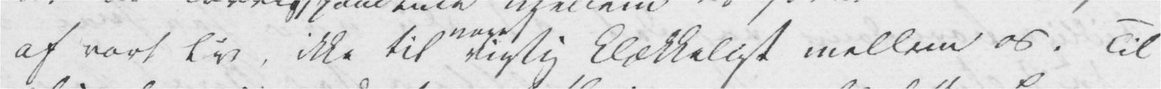af vort Liv, ikke til rigtig Klækkeligt mellem os. Til
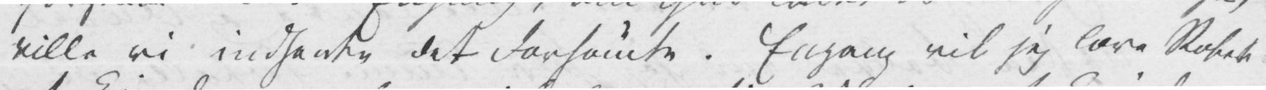ville vi indhente det Forsømte. Engang vil jeg lære Robert
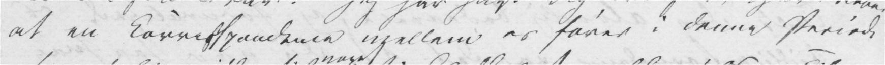at en Korresspondence mellem os fører i denne Periode
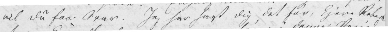vil Du faa Svar. Jeg har sagt Dig det før, kjere Robert
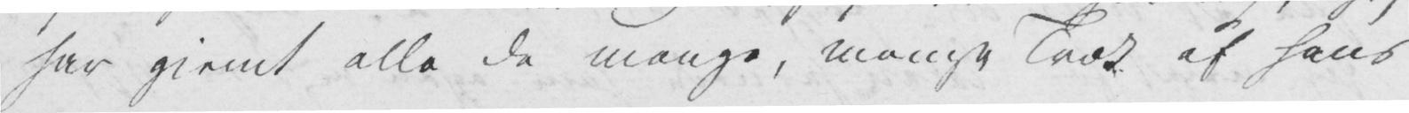har gjemt alle de mange, mange Træk af hans
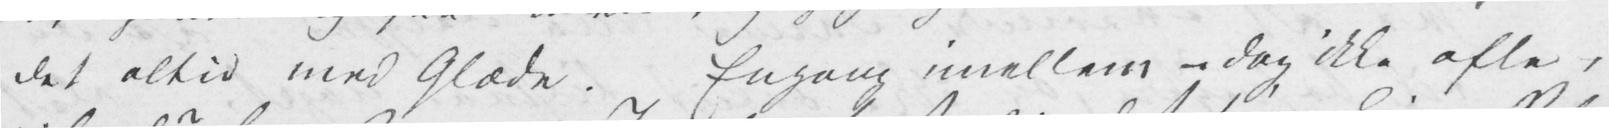det altid med Glæde. Engang imellem – dog ikke ofte,
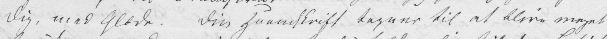Dig, med Glæde. Din Haandskrift tegner til at blive meget
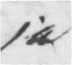ja
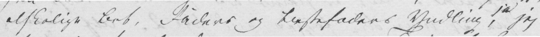elskelige Bob, Faders og Bestefaders Yndling, jeg
